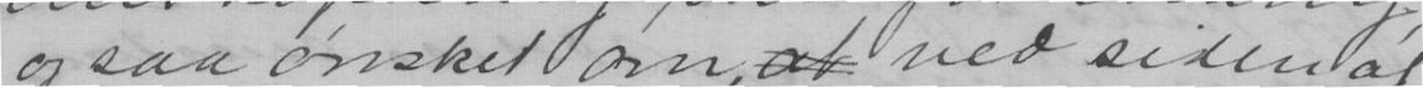og saa ønsket om, at ved siden af
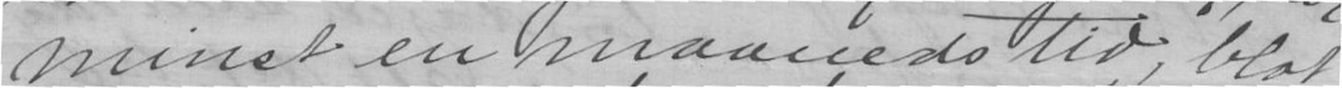minst en maaneds tid, blot
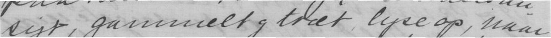sigt, gammelt og træt, lyse op, naar
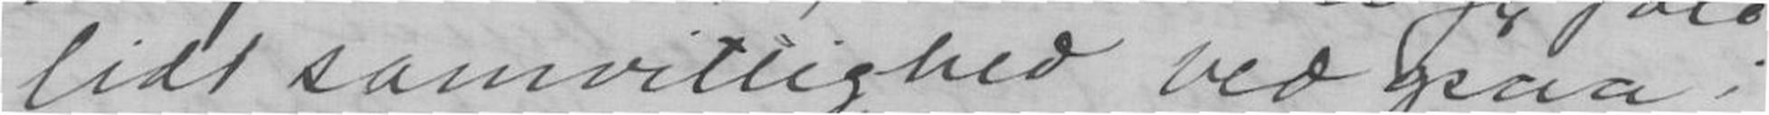lidt samvittighed ved ogsaa i
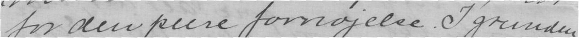for den pure fornöjelse. I grunden
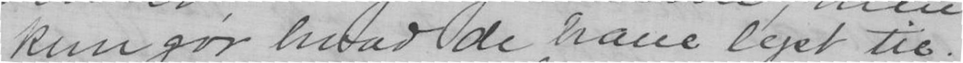kun gör hvad hvad de have lyst til.
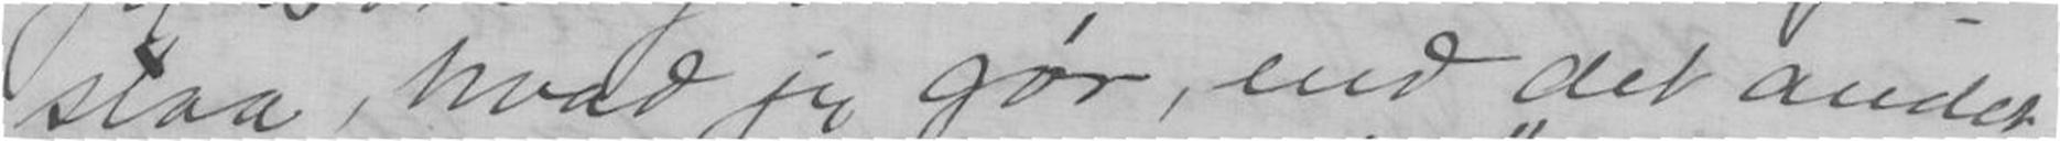staa, hvad jeg gör, end det andet
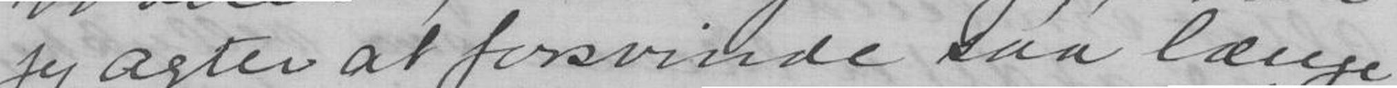jeg agter at forsvinde saa længe
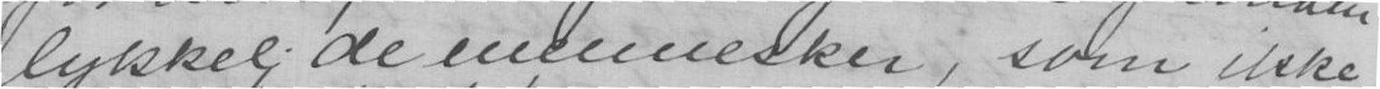lykkelig de mennesker, som ikke
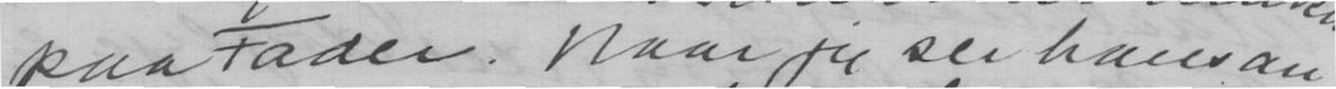paa Fader. Naar jeg ser hans an-
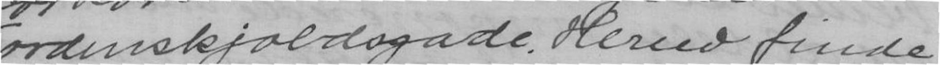Tordenskjoldsgade. Herned finde
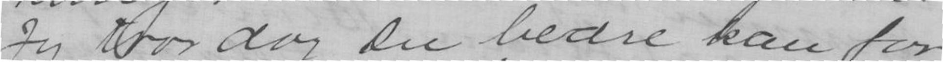Jeg tror dog Du bedre kan for
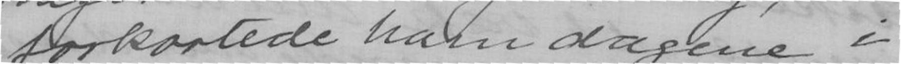forkortede ham dagene i
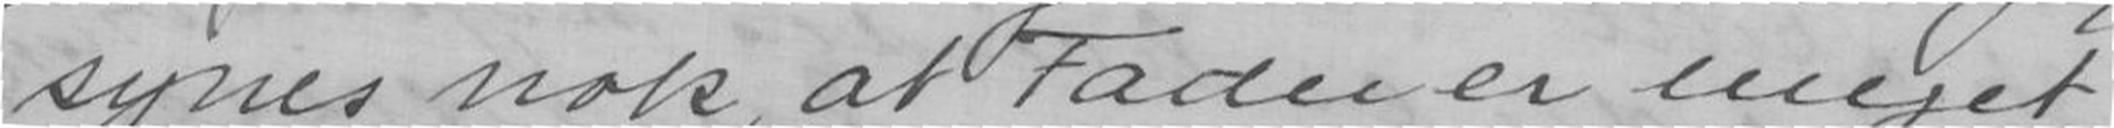synes nok, at Fader er meget
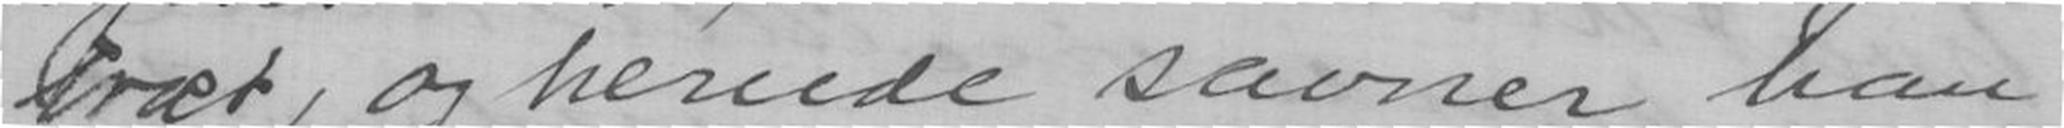træt, og hernede savner han
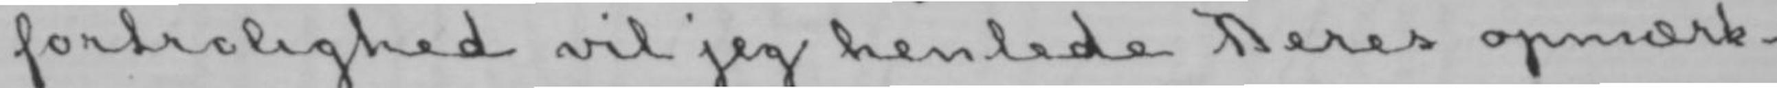fortrolighed vil jeg henlede Deres opmærk-
